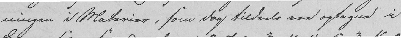ningen i Materier, som dog tildeels ere optagne i
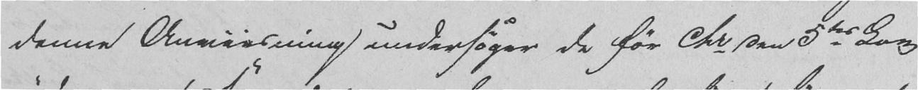denne Anviisning undersøger de før Chr den 5tes Love
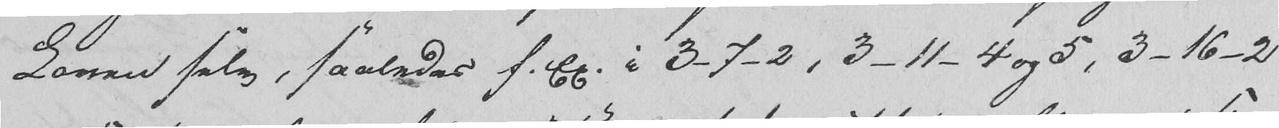Loven selv, saaledes f. Ex. i 3-7-2, 3-11-4 og 5, 3-16-2
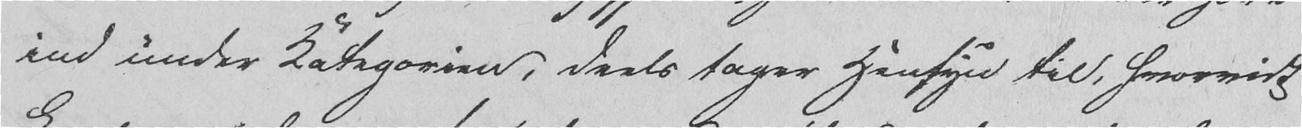ind under Kategorien, deels tager Hensyn til, hvorvidt
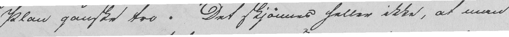Plan ganske tro. Det skjønnes heller ikke, at man
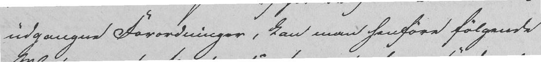udgangne Forordninger, kan man henføre følgende
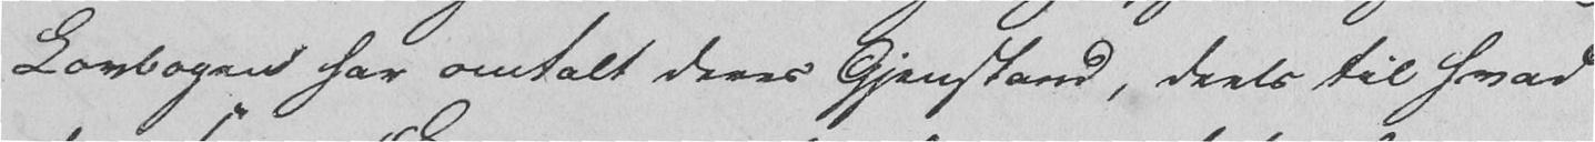Lovbogen har omtalt deres Gjenstand, deels til hvad
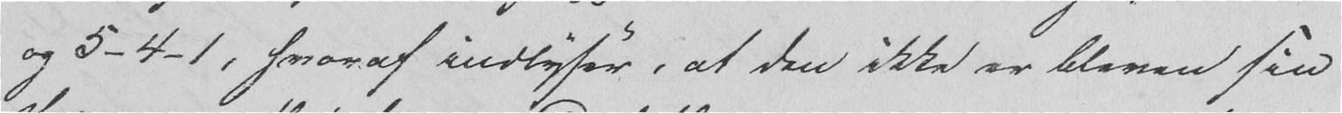og 5-4-1, hvoraf indlyser, at den ikke er bleven sin
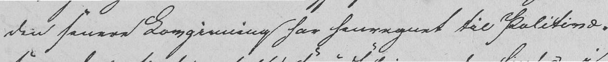den senere Lovgivning har henregnet til Politivæ-
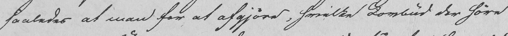saaledes at man for at afgjøre, hvilke Lovbud der høre
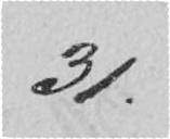31.
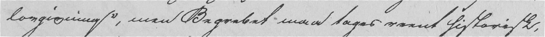lovgivning", men Begrebet maa tages reent historisk,
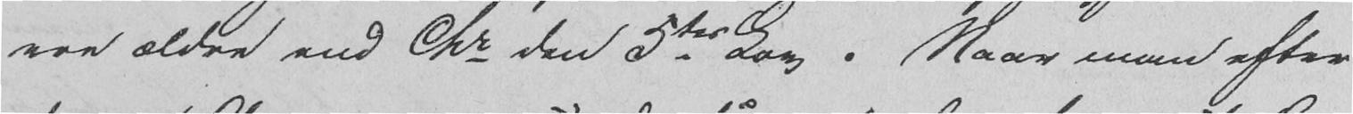ere ældre end Chr den 5tes Lov. Naar man efter
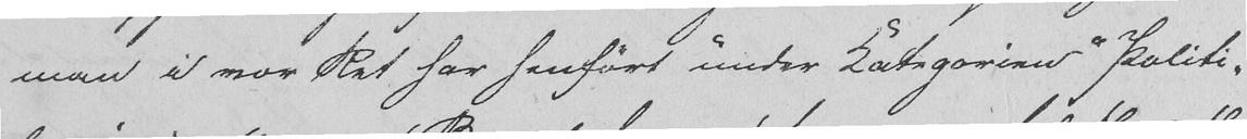man i vor Ret har henført under Kategorien "Politi-
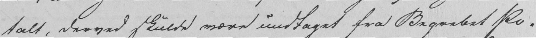talt, derved skulde være undtaget fra Begrebet Po-
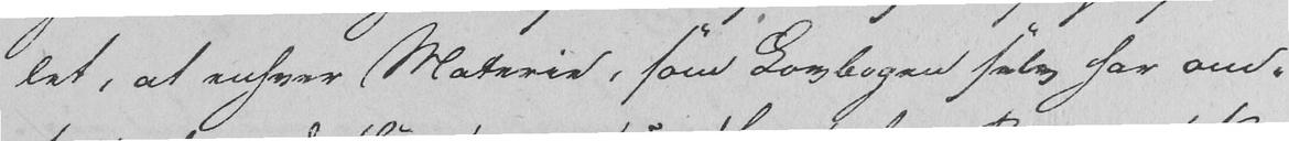let, at enhver Materie, som Lovbogen selv har om-
